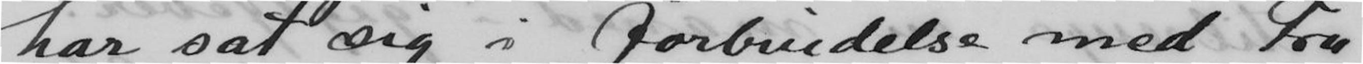har sat sig i forbindelse med Fru
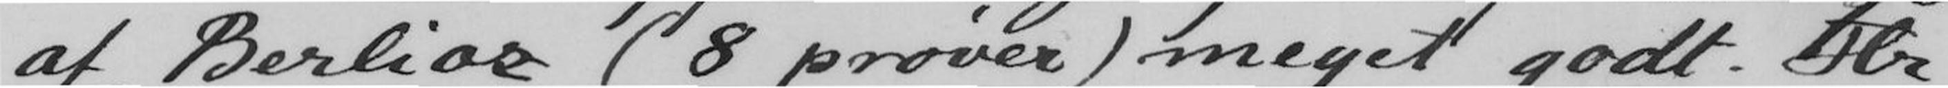af Berlioz (8 prøver) meget godt. Hr.
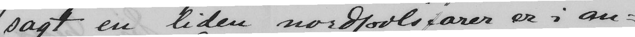sagt en liden nordpolsfarer er i an-
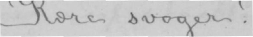Kære svoger!
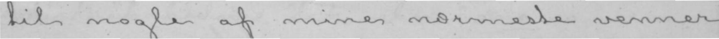til nogle af mine nærmeste venner
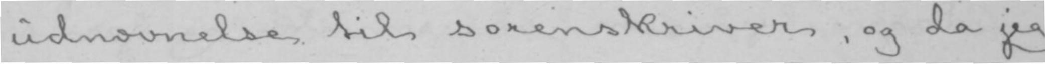udnævnelse til sorenskriver, og da jeg
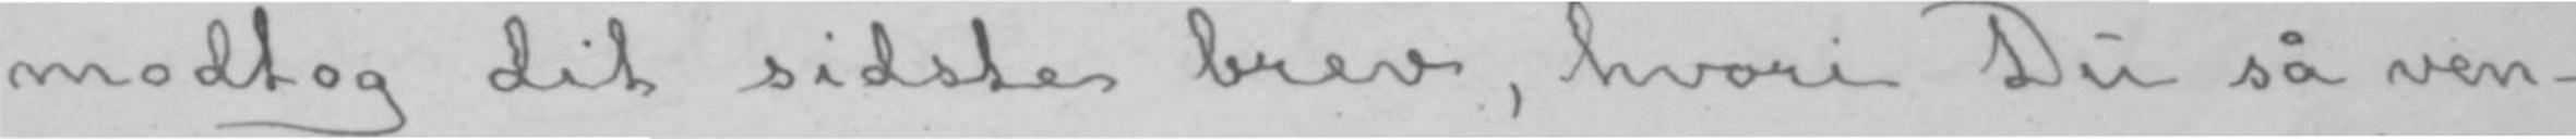modtog dit sidste brev, hvori Du så ven-
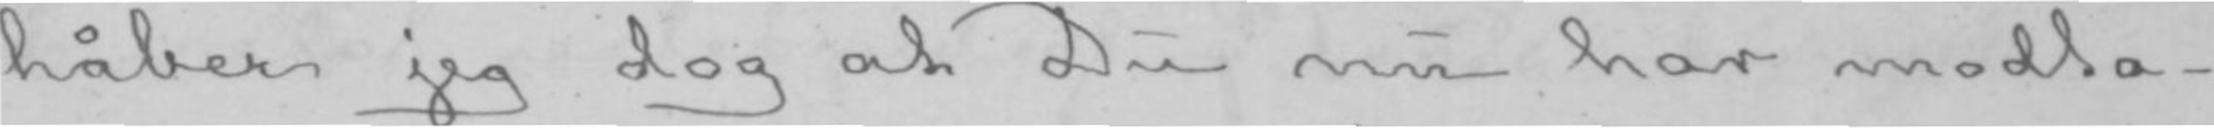håber jeg dog at Du nu har modta-
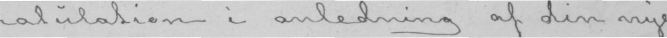gratulation i anledning af din nye
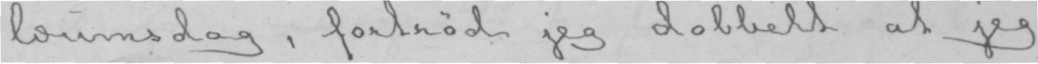læumsdag, fortrød jeg dobbelt at jeg
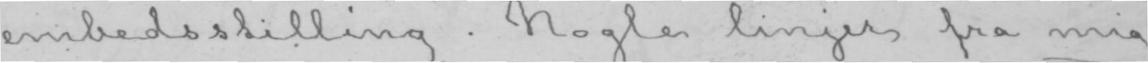embedsstilling. Nogle linjer fra mig
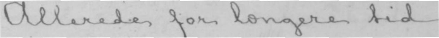Allerede for længere tid
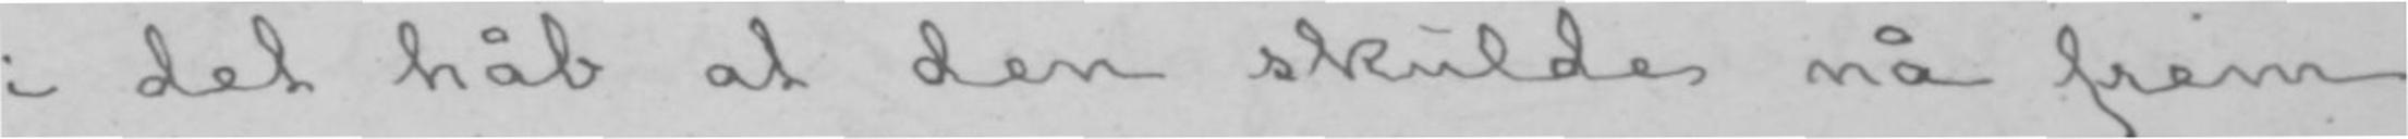i det håb at den skulde nå frem
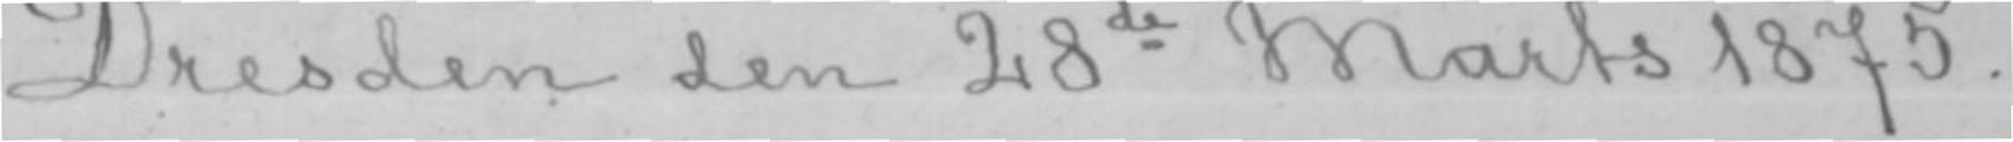Dresden den 28de Marts 1875.
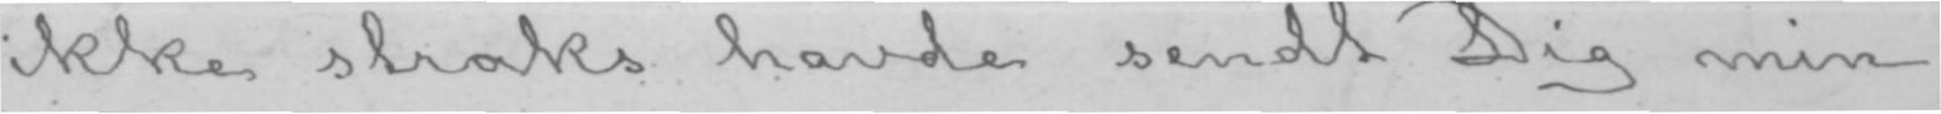ikke straks havde sendt Dig min
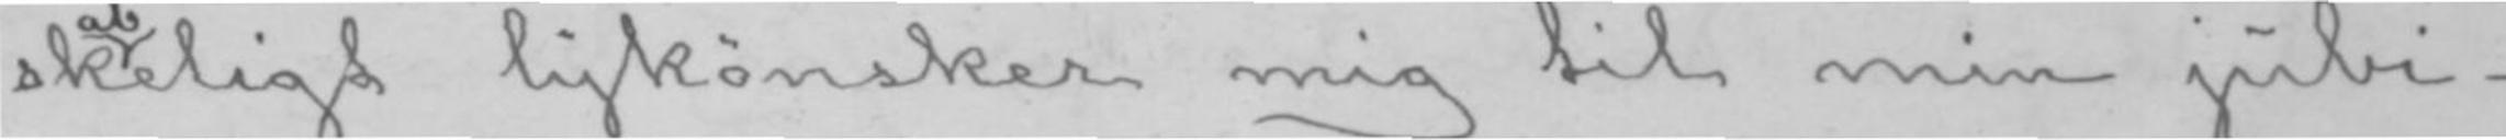skabeligt lykønsker mig til min jubi-
